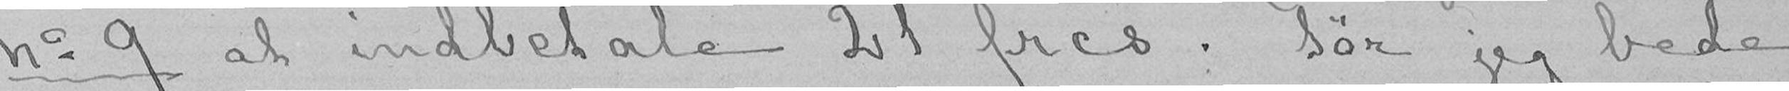No 9 at indbetale 21 frcs. Tør jeg bede
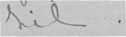til.
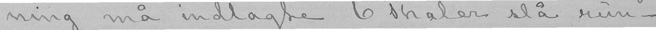ning må indlagte 6 Thaler slå run-
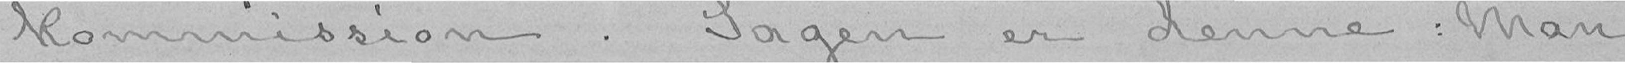kommission. Sagen er denne: Man
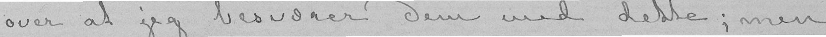over at jeg besværer Dem med dette; men
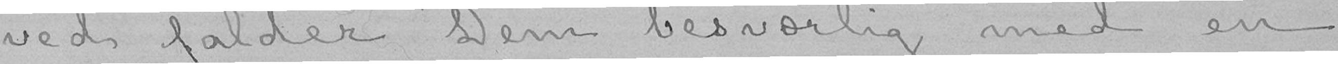ved falder Dem besværlig med en
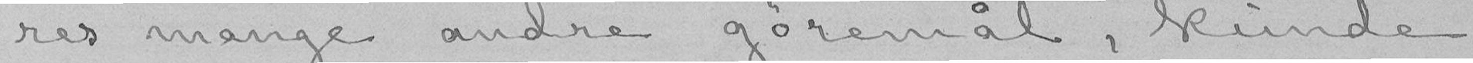res mange andre gøremål, kunde
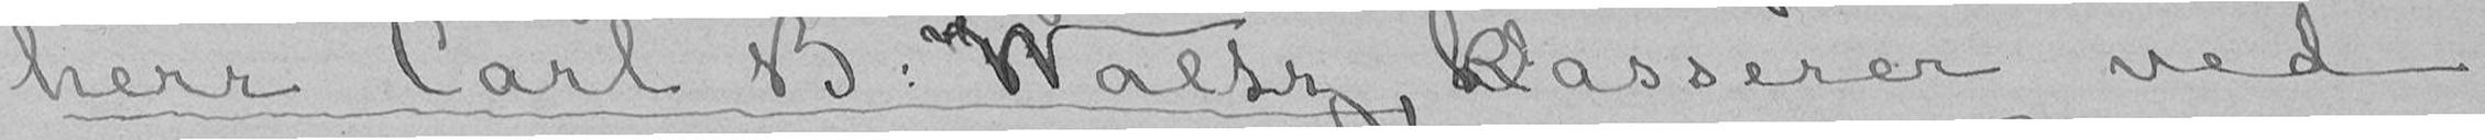herr Carl B: Waltz, kasserer ved
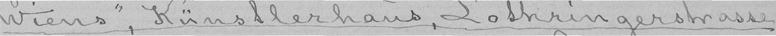Wiens», Künstlerhaus, Lothringerstrasse,
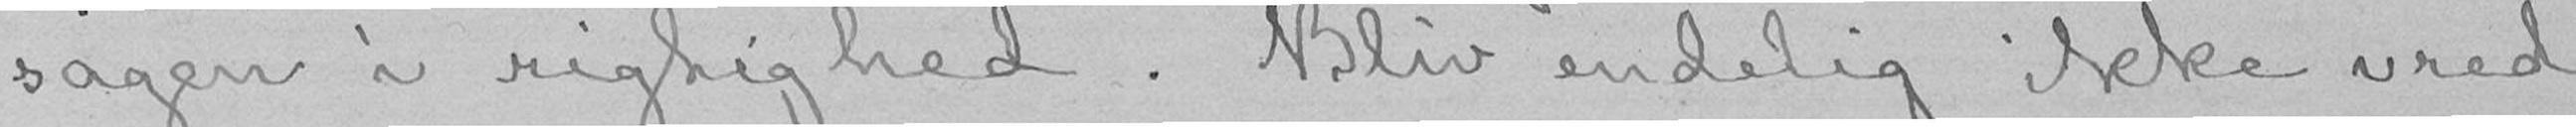sagen i rigtighed. Bliv endelig ikke vred
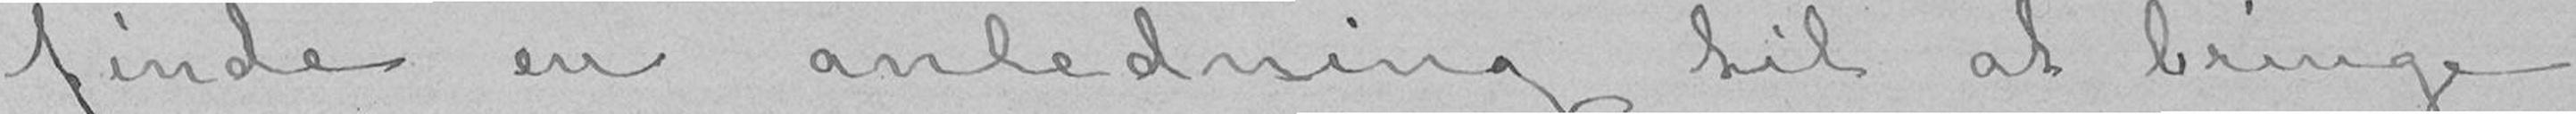finde en anledning til at bringe
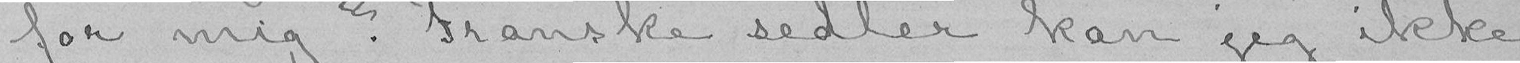for mig? Franske sedler kan jeg ikke
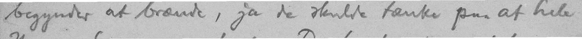begynder at brænde, ja de skulde tænke paa at hele
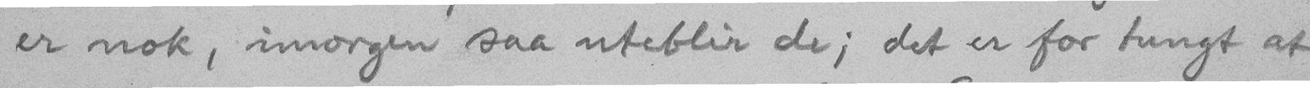er nok, imorgen saa uteblir de; det er for tungt at
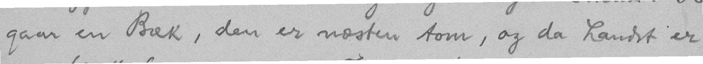gaar en Bæk, den er næsten tom, og da Landet er
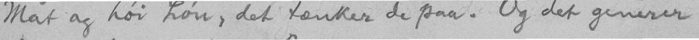Mat og høi Løn, det tænker de paa. Og det generer
